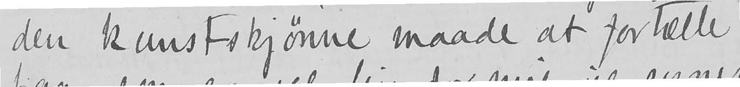den kunstskjønne maade at fortælle
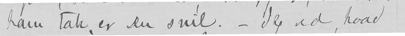ham tak, er Du snil. - Jeg ved, hvad
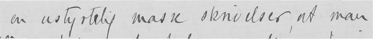en ustyrtelig masse skrivelser, at man
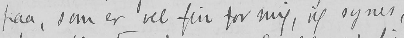paa, som er vel fin for mig, jeg synes
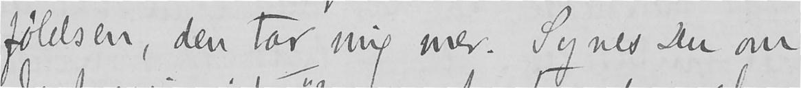følelsen, den tar mig mer. Synes Du om
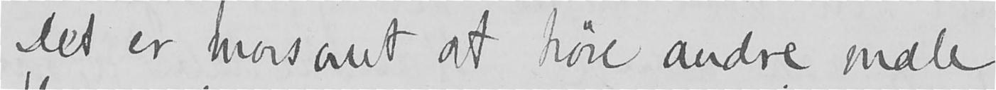Det er morsomt at høre andre male
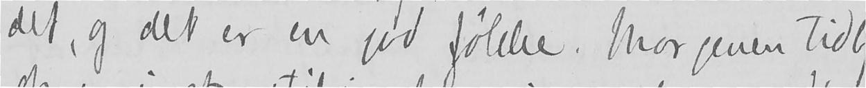det, og det er en god følelse. Morgenen tidlig
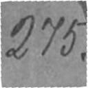275.
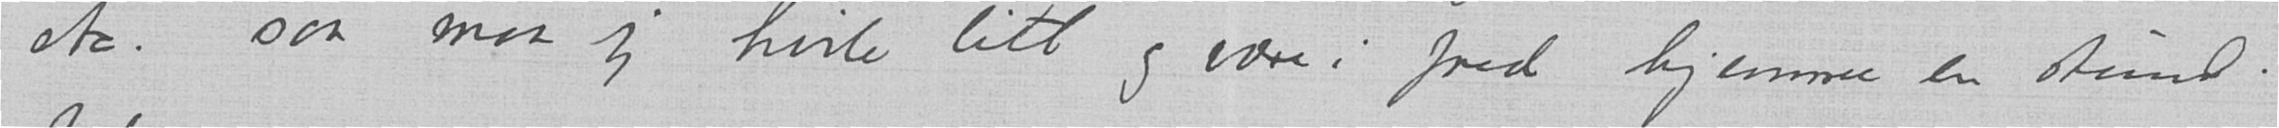etc. saa maa jeg hvile litt og være i fred hjemme en stund.
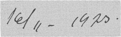16/11 -1923.
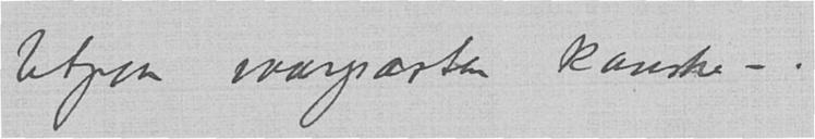Utpaa vaarparten kanske -.
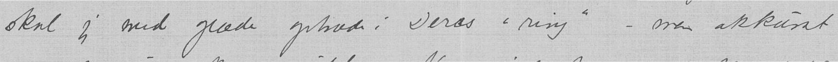skal jeg med glæde optræde i Deres "ring" – men akkurat
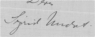Sigrid Undset.
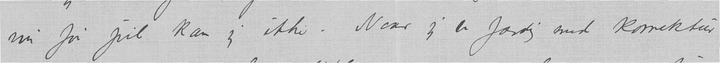nu før jul kan jeg ikke. Naar jeg er færdig med korrektur
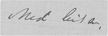Med hilsen,
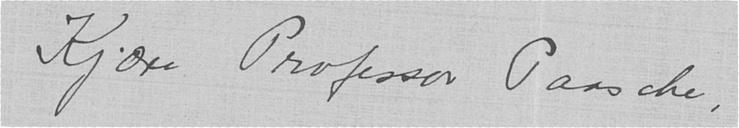Kjære Professor Paasche,
